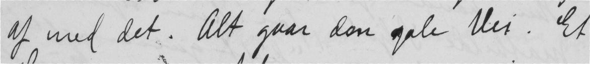af med det. Alt gaar den gale Vei. Et
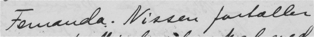Fenanda Nissen fortæller
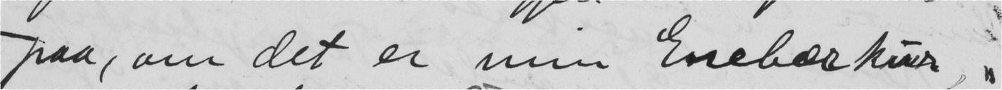paa, om det er min Enebærkur
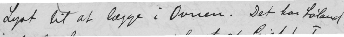Lyst til at lægge i Ovnen. Det har Løland
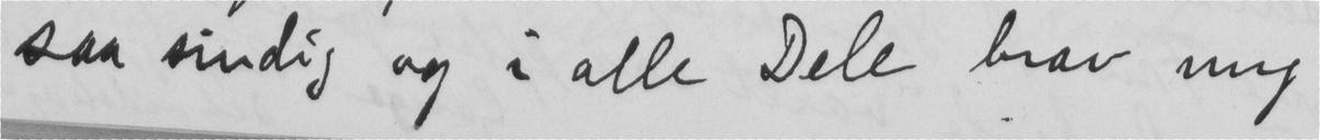saa sindig og i alle Dele brav mig
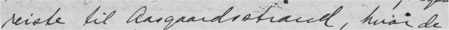reiste til Aasgaardsstrand, hvor de
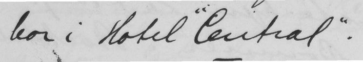bor i Hotel "Central".
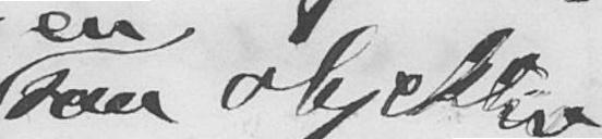saa objektiv
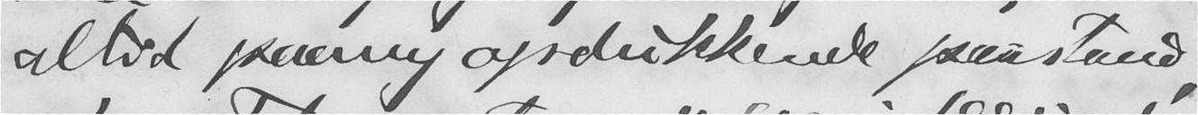altid paany opdukkende paastand,
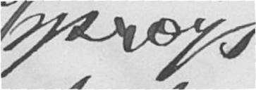sprogs
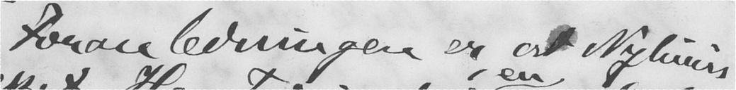Foranledningen er, at Nyhuus
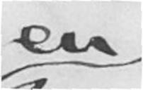en
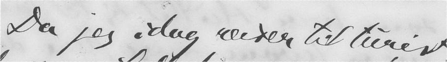Da jeg idag reiser til turist-
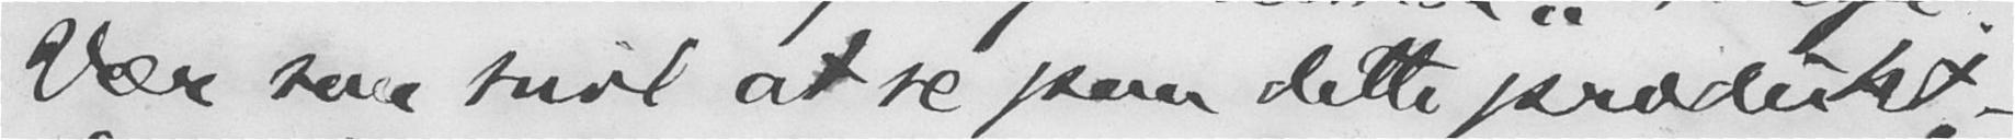Vær saa snil at se paa dette produkt, -
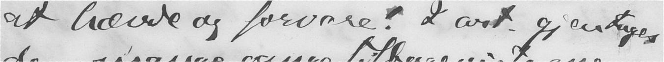at hævde og forvare! I art. gjentages
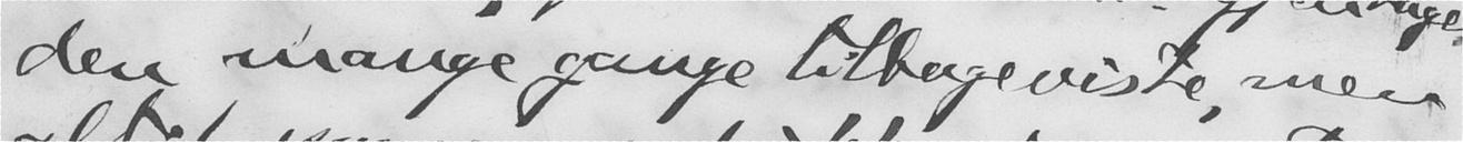den mange gange tilbageviste, men
